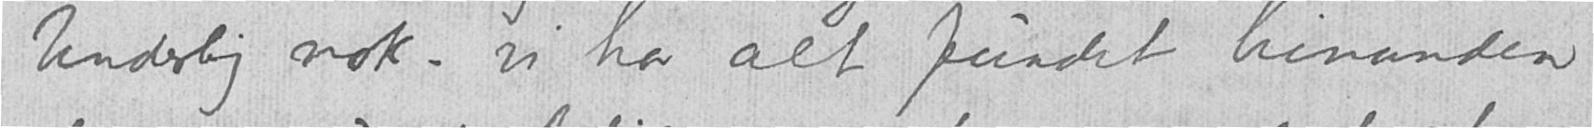Underlig nok - vi har alt fundet hinanden
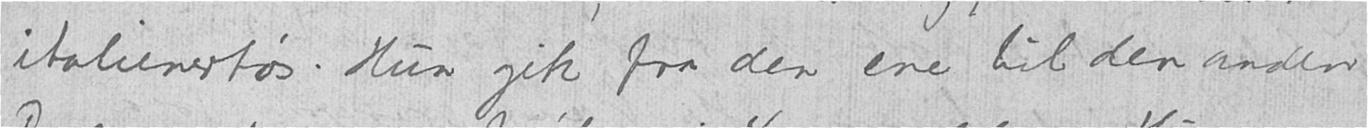italienertøs. Hun gik fra den ene til den anden.
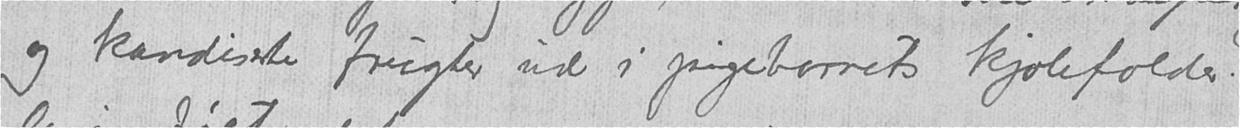og kandiserte frugter ud i pigebarnets kjolefolder.
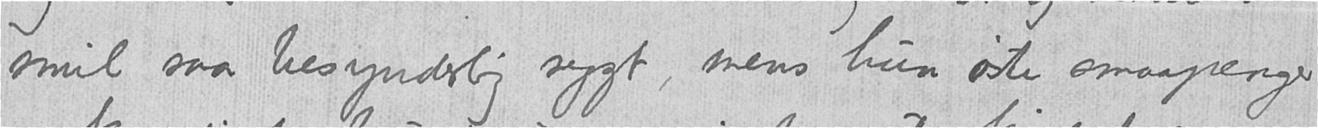smil saa besynderlig sygt, mens hun øste smaapenger
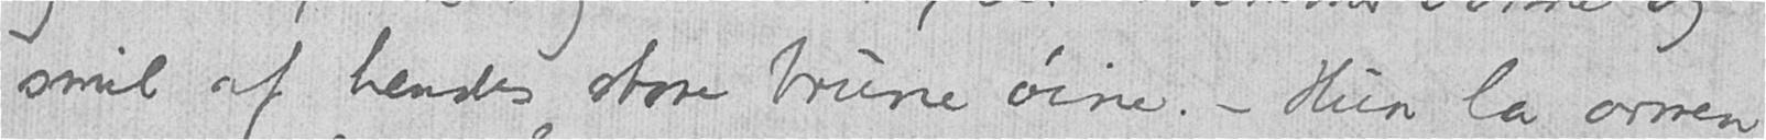smil af hendes store brune øine. - Hun la armen
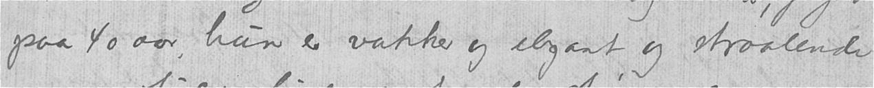paa 40 aar, hun er vakker og elegant og straalende
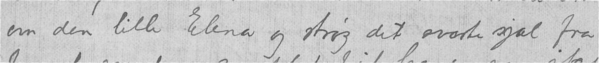om den lille Elena og strøg det svarte sjal fra
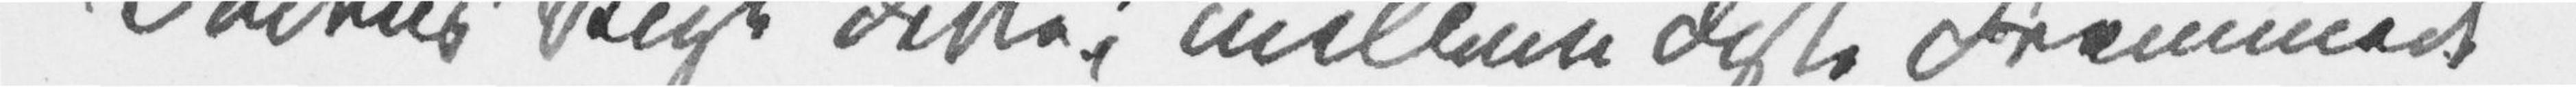Dødens Rige dette! mellem disse Fremmede
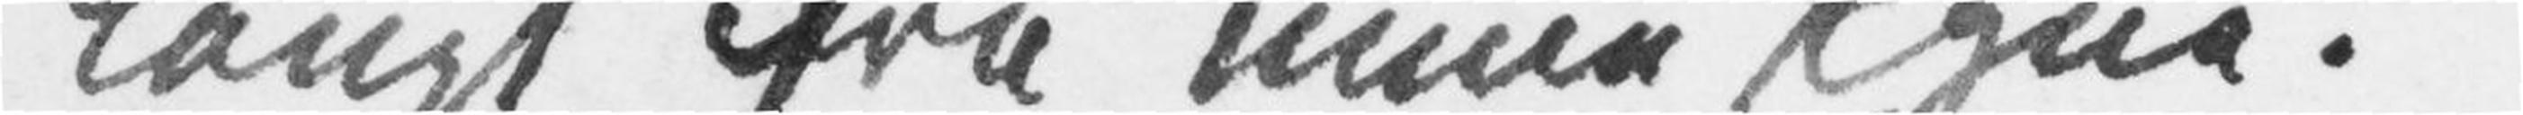langt fra mine Egne!
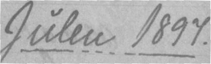Julen 1897.
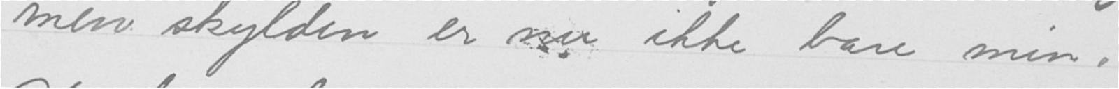men skylden er nu ikke bare min.
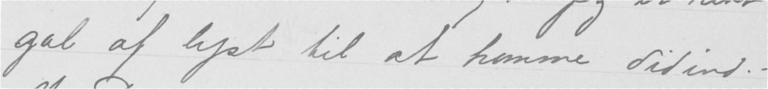gal af lyst til at komme did ind. -
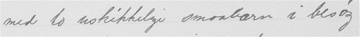med to uskikkelige smaabarn i besøg
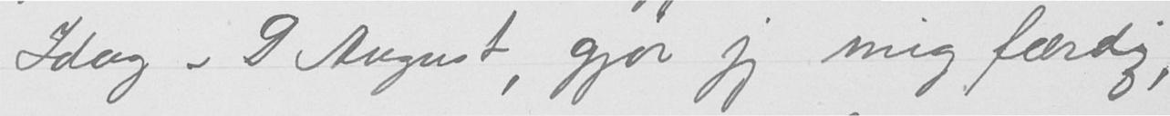Idag - 9. August, gjør jeg mig færdig,
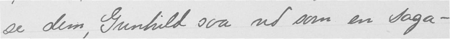se dem, Gunhild saa ud som en saga-
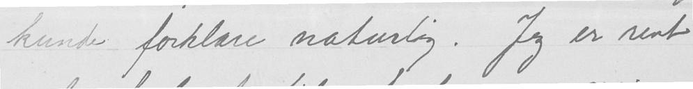kunde forklare naturlig. Jeg er rent
